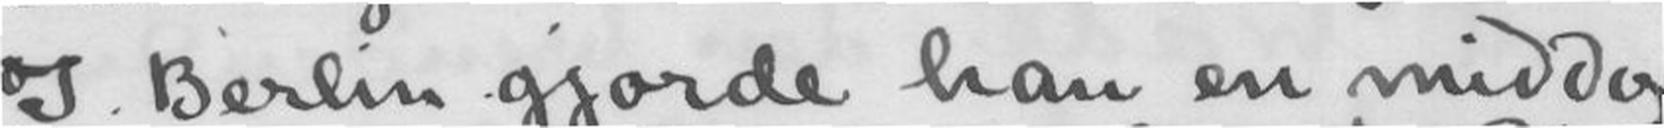I Berlin gjorde han en middag
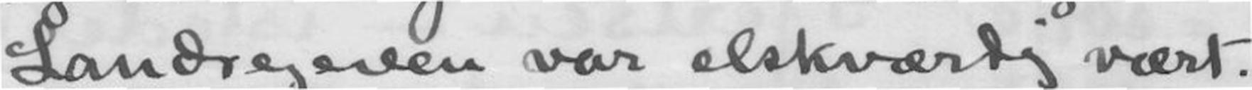Landgreven var elskværdig vært.
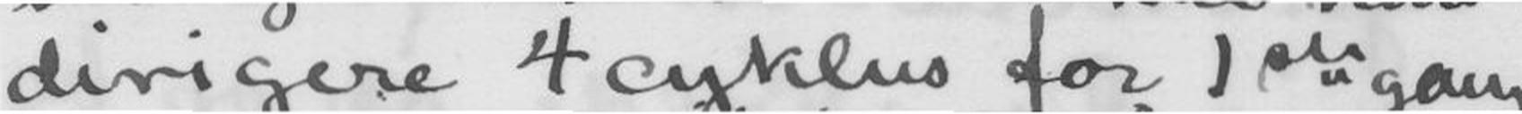dirigere 4 cyklus for 1ste gang
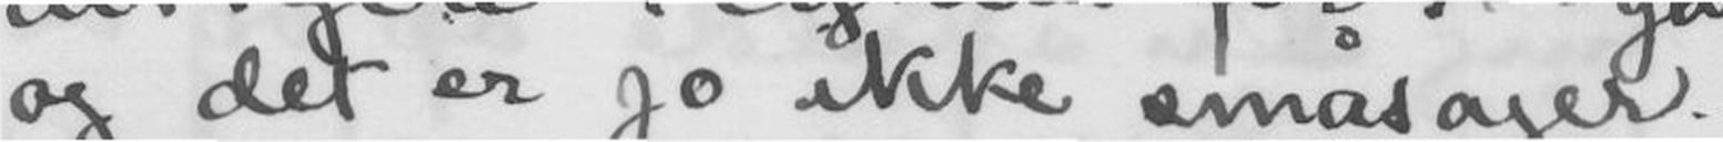og det er jo ikke småsager. -
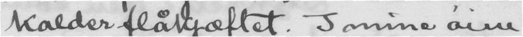kalder flåkjæftet. I mine øine
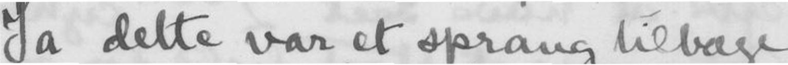Ja dette var et sprang tilbage
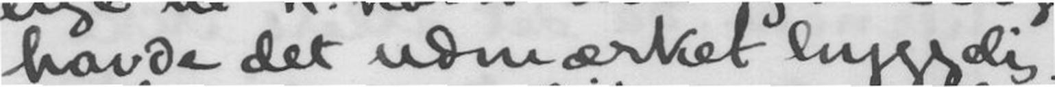havde det udmærket hyggelig.
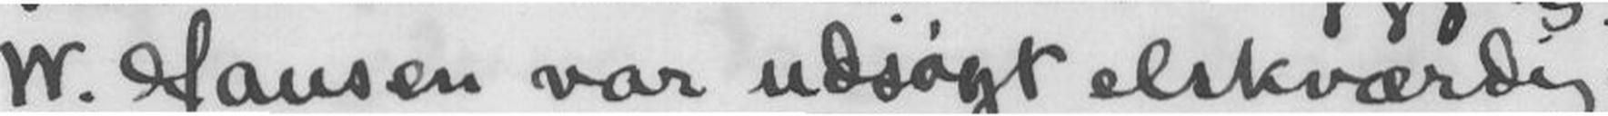W. Hansen var udsøgt elskværdig.
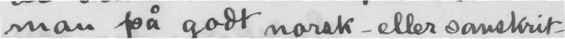man på godt norsk - eller sanskrit -
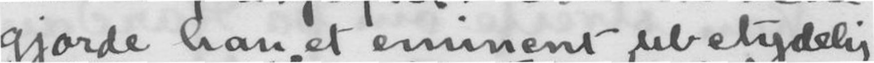gjorde han et eminent ubetydelig
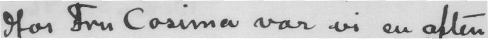Hos Fru Cosima var vi en aften
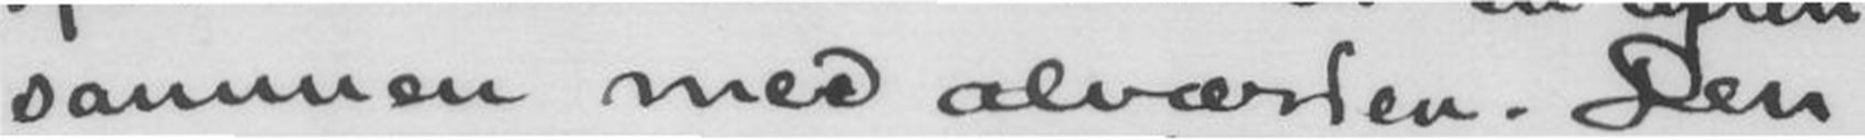sammen med alværden. Den
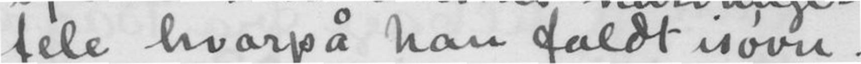fele hvorpå han faldt isøvn.
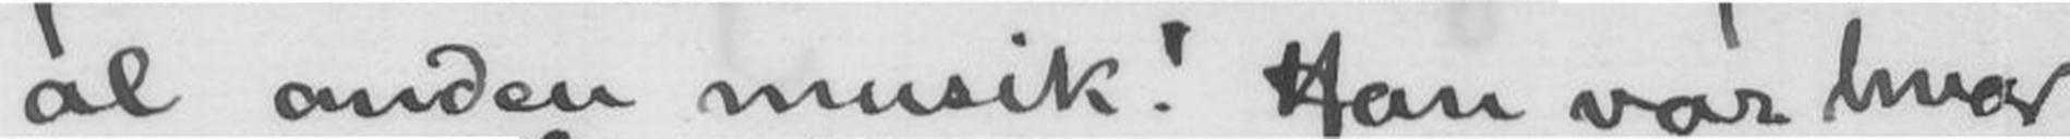al anden musik! Han var hvad
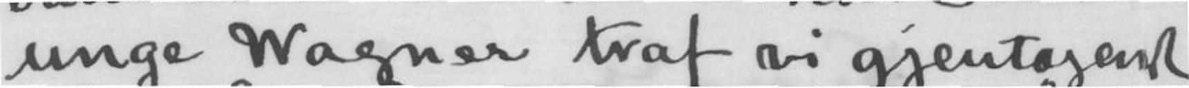unge Wagner traf vi gjentagende
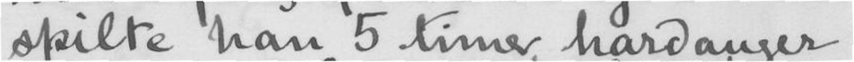spilte han 5 timer hardanger-
